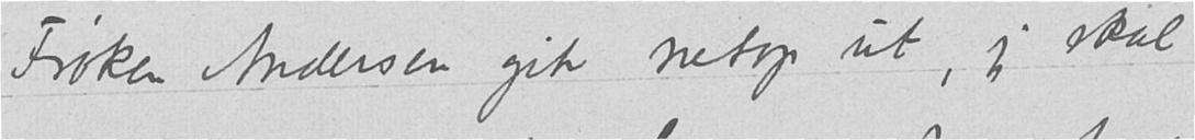Frøken Andersen gik netop ut, jeg skal
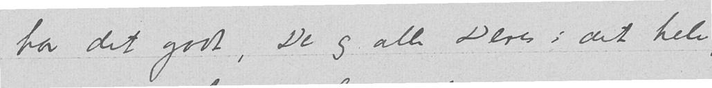har det godt, De og alle Deres i det hele,
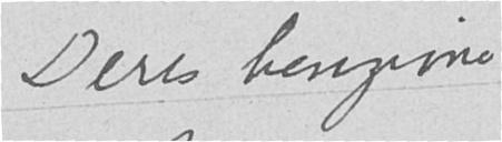Deres hengivne
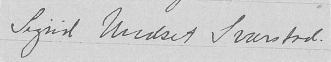Sigrid Undset Svarstad.
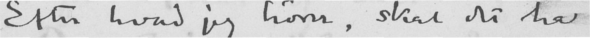Efter hvad jeg hører, skal det ha
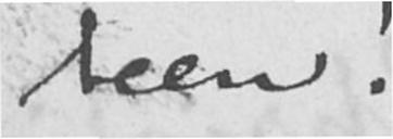teen!
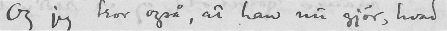Og jeg tror også, at han nu gjør, hvad
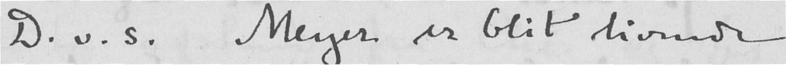D.v.s. Meyer er blit livende
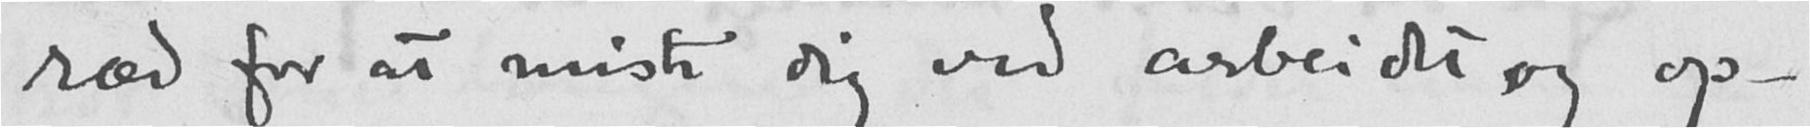ræd for at miste dig ved arbeidet og op-
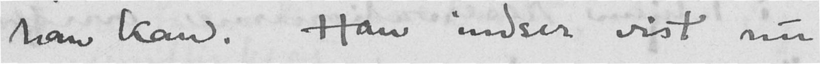han kan. Han indser vist nu
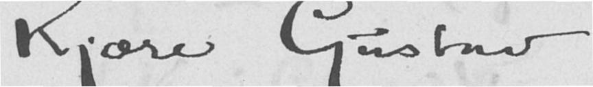Kjære Gustav
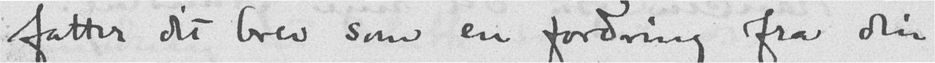fatter dit brev som en fordring fra din
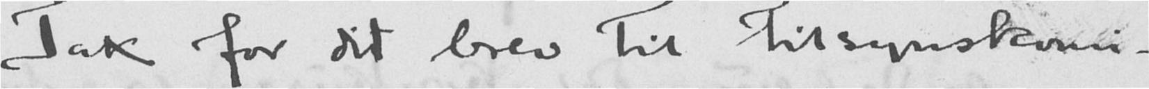Tak for dit brev til tilsynskomi-
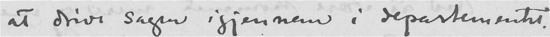at drive sagen igjennem i departementet.
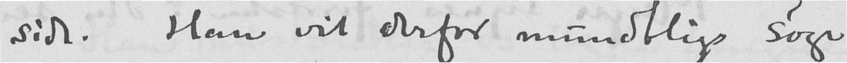side. Han vil derfor mundtlig søge
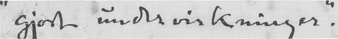"gjort undervirkninger".
